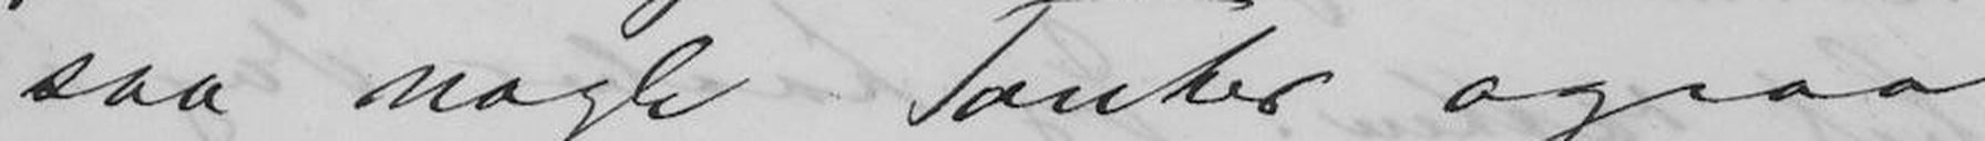saa nogle Tanker ogsaa
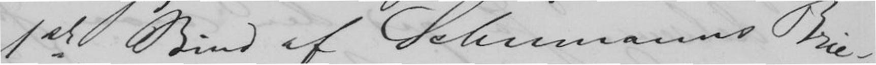1ste Bind af Schumanns Brie-
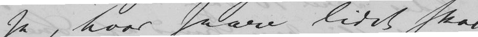Ja, hvor saare lidet skal
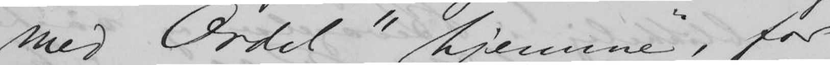med Ordet "hjemme", for
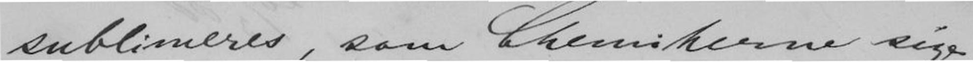sublimeres, som Chemikerne sige
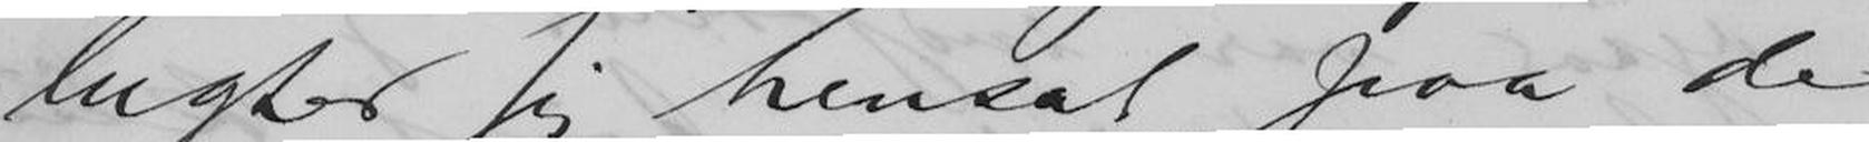lugter sig hensat paa de
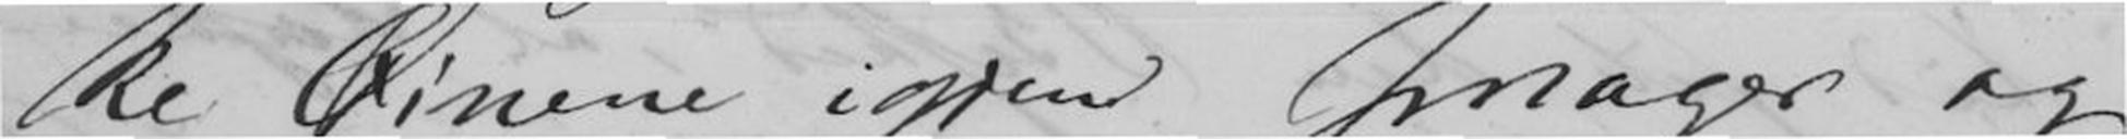ke Øinene igjen smager og
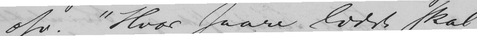osv. "Hvor saare lidet skal
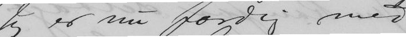Jeg er nu færdig med
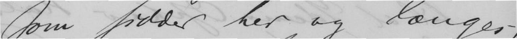som sidder her og længes!
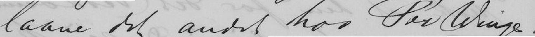laane det andet hos Per Winge.
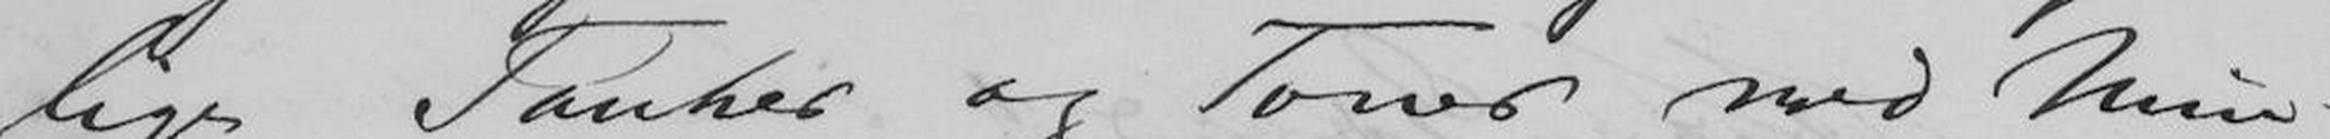lige Tanker og Toner med Min-
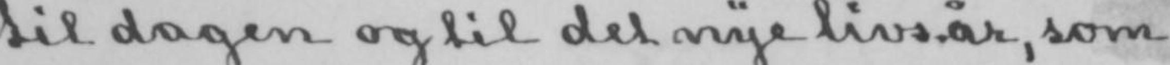til dagen og til det nye livs-år, som
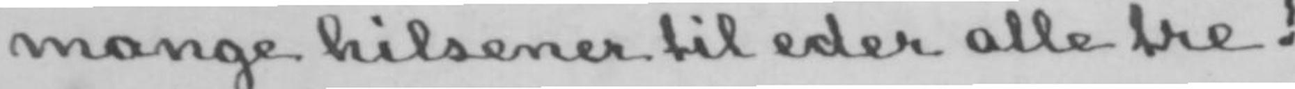mange hilsener til eder alle tre!
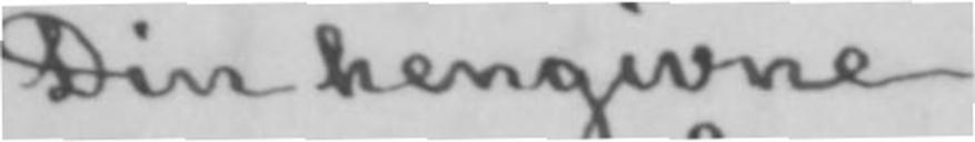Din hengivne
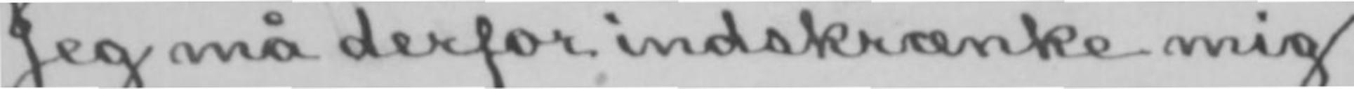Jeg må derfor indskrænke mig
